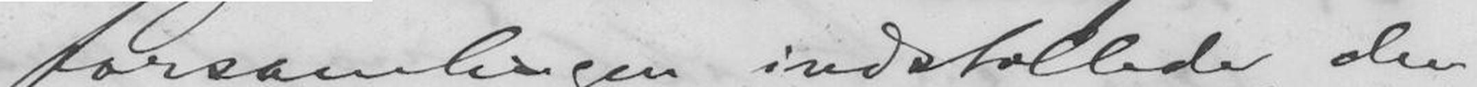forsamlingen indstillede den
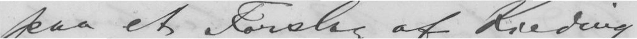paa et Forslag af Kinding
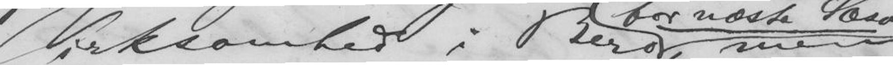Virksomhed i Bero for, men
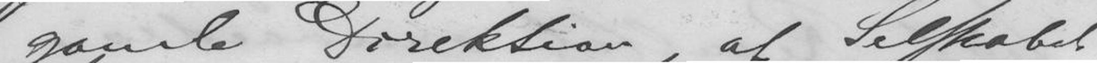gamle Direktion, at Selskabet
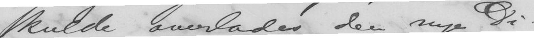skulde overlades den nye Di-
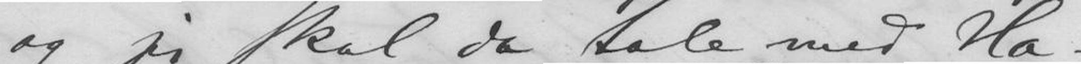og jeg skal da tale med Ha-
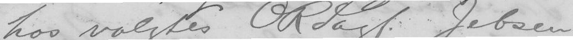hos valgtes OR Sagf Jebsen
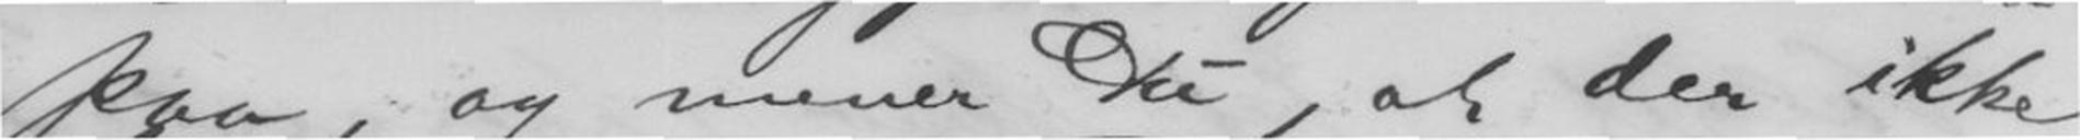paa, og mener Du, at der ikke
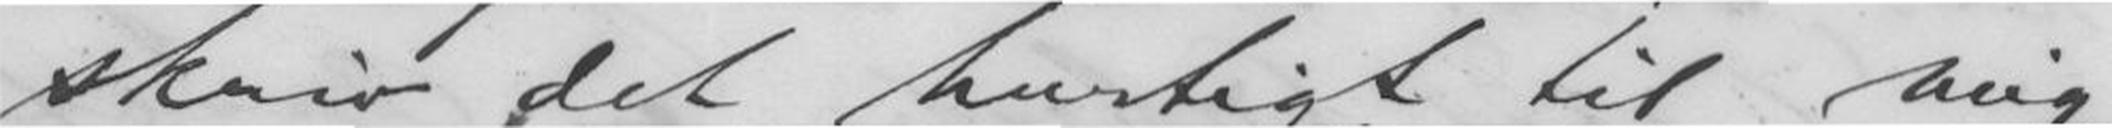skriv det hurtigt til mig
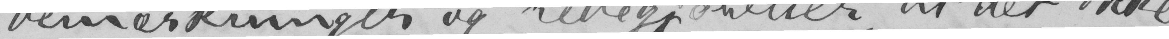bemærkninger og redegjørelser, at det ikke
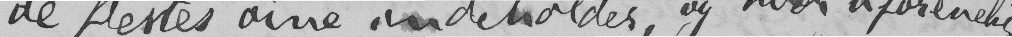de flestes øine indeholder, og hvor uforenelig,
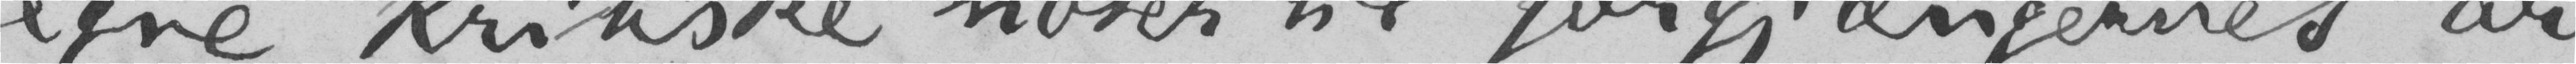egne kritiske noter til forgjængernes ar-
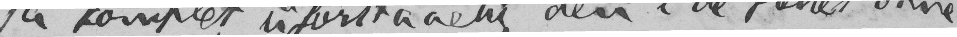ja komplet uforstaaelig den i de flestes øine
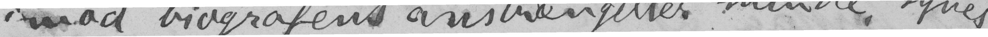imod biografens anstrængelser minde, synes
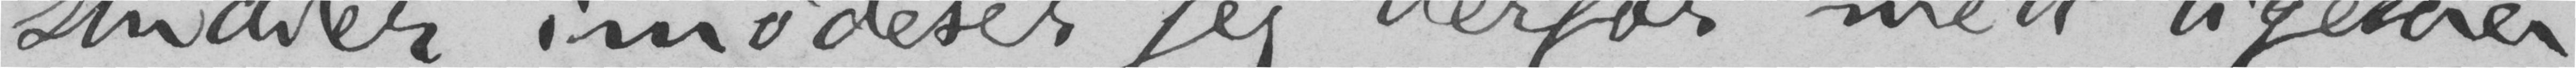studier imødeser jeg derfor med ligesaa
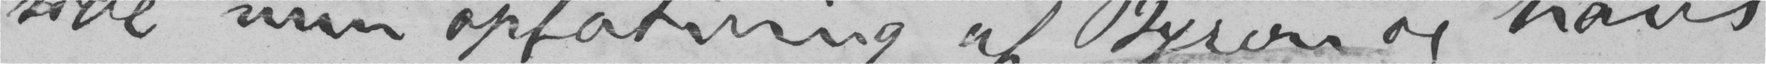side min opfatning af Byron og hans
